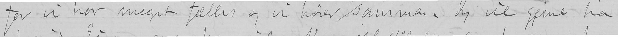for vi har meget fælles og vi hører sammen. Jeg vil gjerne ha
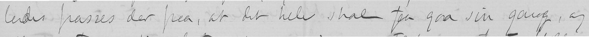ledes passes der paa, at det hele skal faa gaa sin gang, og
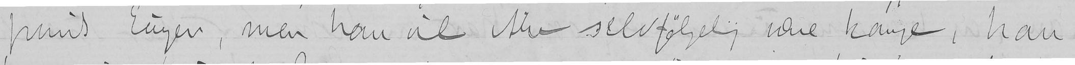prinds Eugen, men han vil ikke selvfølgelig være konge, han
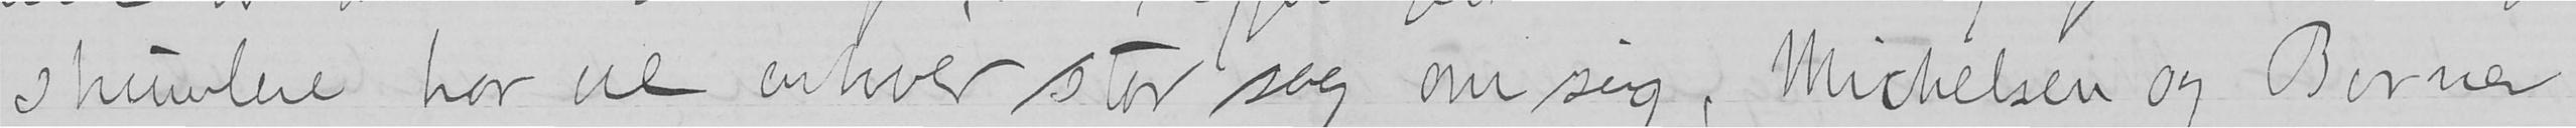skumlere har vel enhver stor sag om sig, Michelsen og Berner
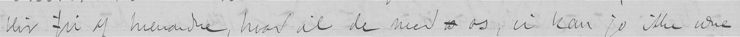blir fri af hverandre, hvad vil de med is os, vi kan jo ikke være
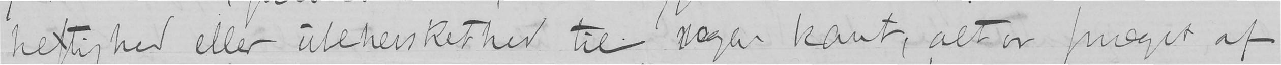heftighed eller ubeherskethed til nogen kant, alt er preget af
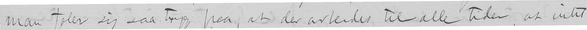man føler sig saa tryg paa, at der arbeides til alle tider, at intet
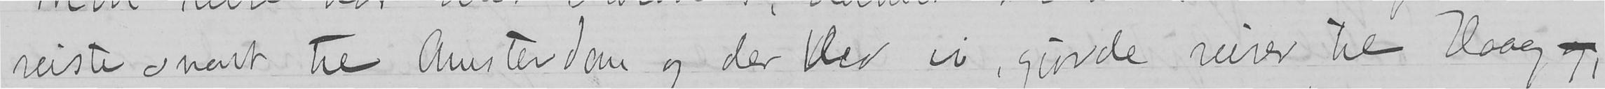reiste snart til Amsterdam og der blev vi, gjorde reiser til Haag og,
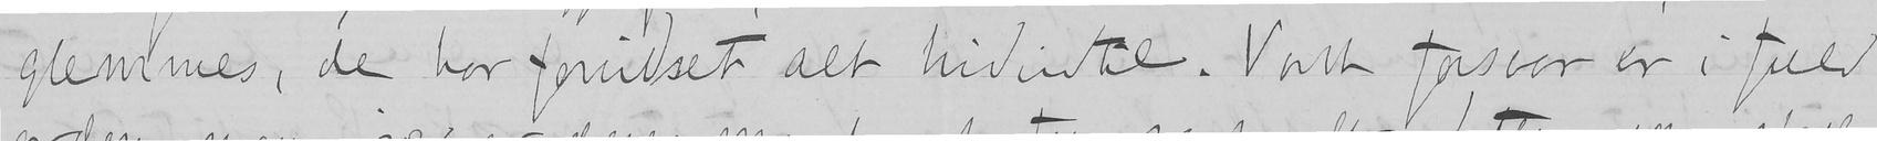glemmes, de har forvidset alt hidindtil. Vort forsvar er i fuld
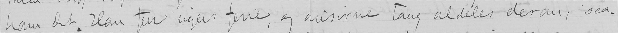ham det. Han faar ingen ferie, og aviserne taug aldeles derom, saa-
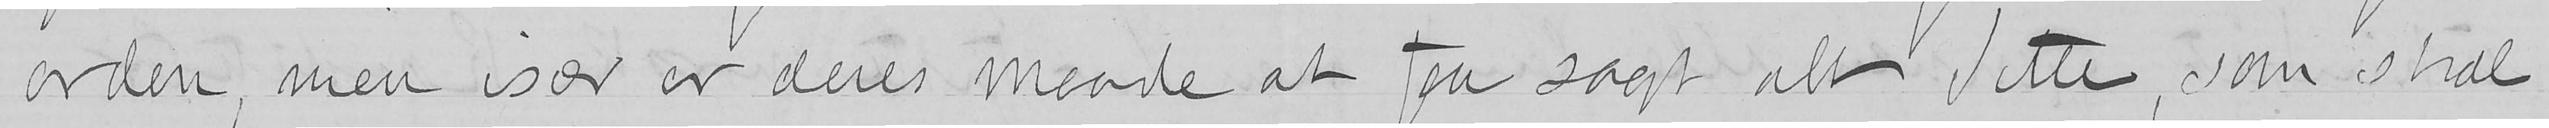orden, men især er deres maade at faa sagt alt dette, som skal
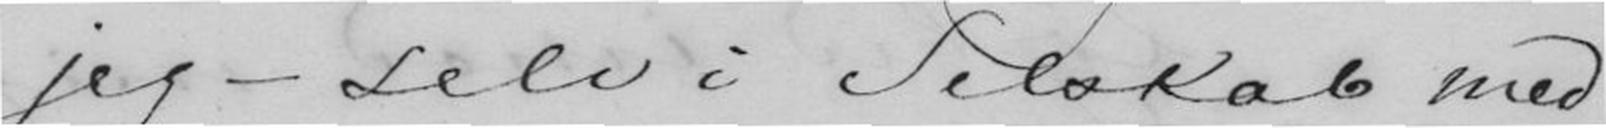jeg - selv i Selskab med
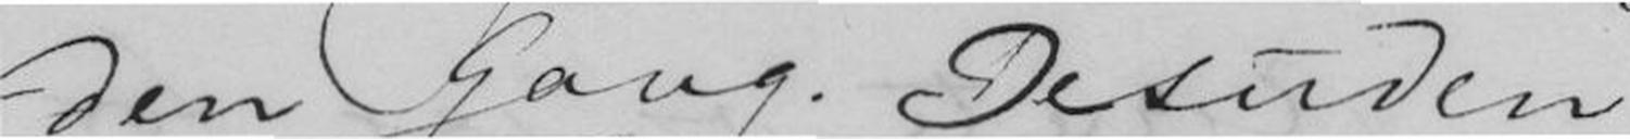den Gang. Desuden
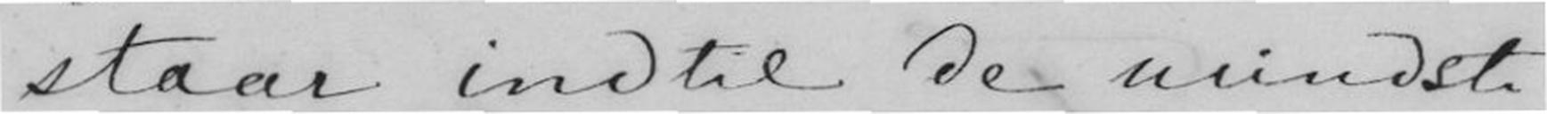staar indtil de mindste
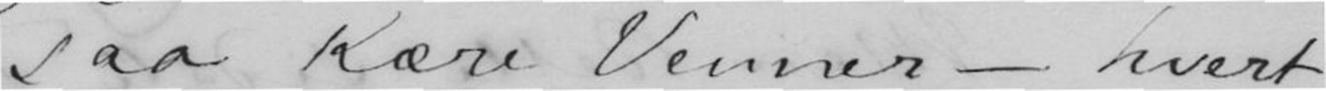saa Kære Venner - hvert
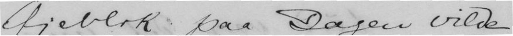Øjeblik paa Dagen vilde
